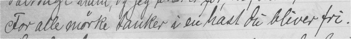For alle mørke tanker i en hast du bliver fri.
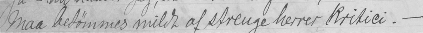Maa bedømmes mildt af strenge herrer kritici. -
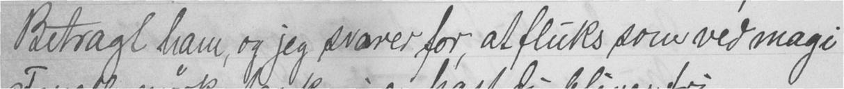Betragt ham, og jeg svarer for, at fluks som ved magi
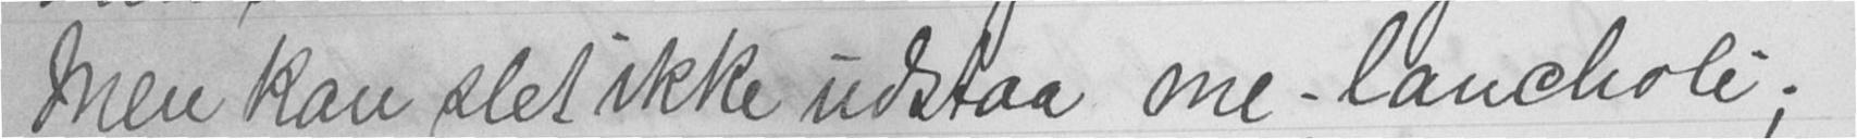Men kan slet ikke udstaa me-lancholi;
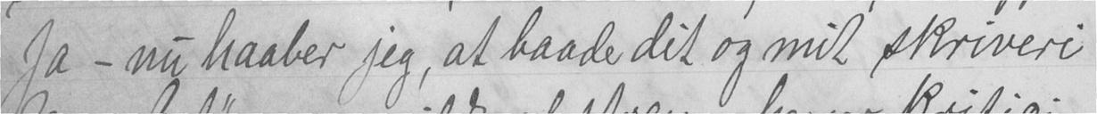Ja - nu haaber jeg, at baade det og mit skriveri
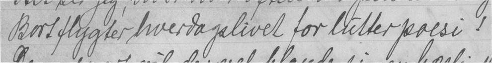Bort flygter hverdagslivet for lutter poesi!
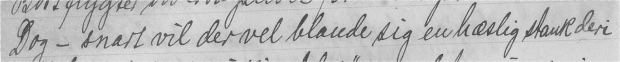Dog - snart vil der vel blande sig en hæslig stank deri
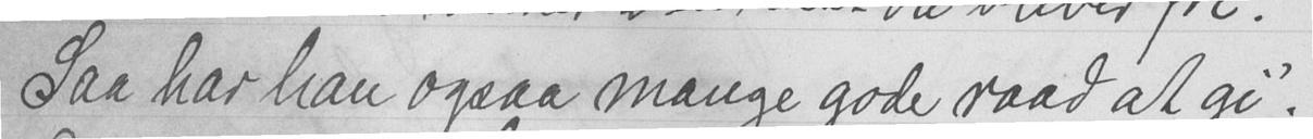Saa har han ogsaa mange gode raad at gi';
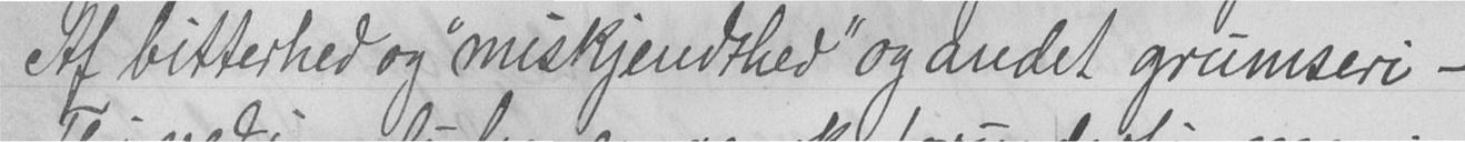Af bitterhed og "miskjendthed" og andet grumseri -
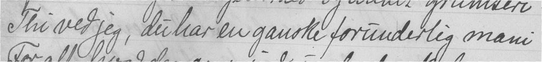Thi ved jeg, du har en ganske forunderlig mani
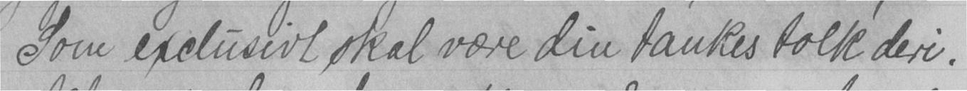Som exclusivt skal være din tankes tolk deri.
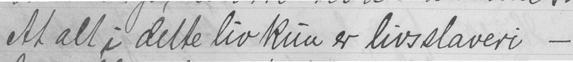At alt i dette liv kun er livsslaveri -
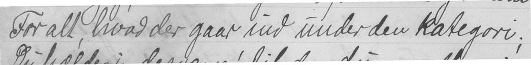For alt, hvad der gaar ind under den kategori;
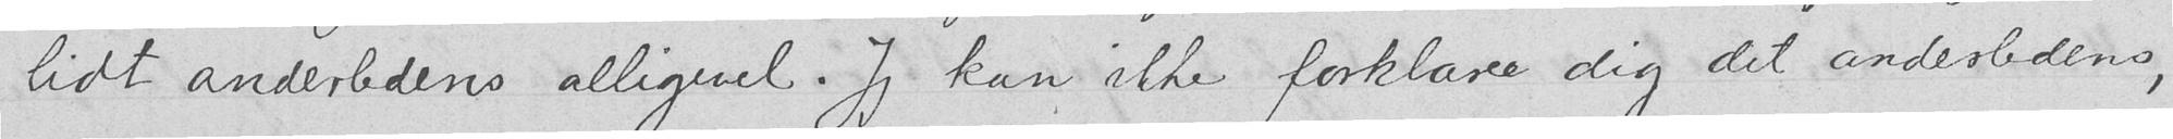lidt anderledens alligevel. Jeg kan ikke forklare dig det andesledens,
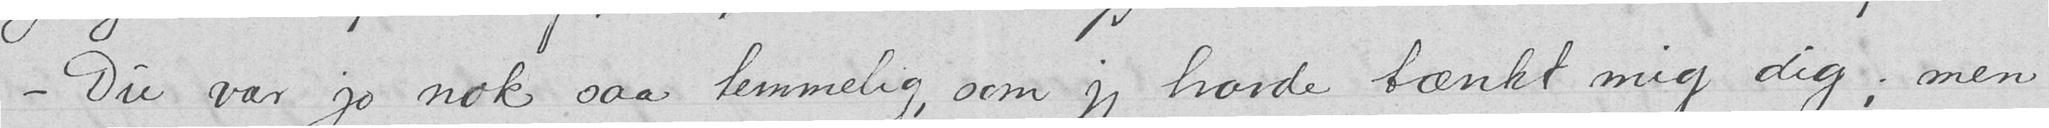- Du var jo nok saa temmelig, som jeg havde tænkt mig dig; men
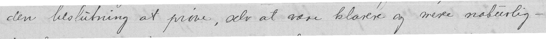den beslutning at prøve, selv at være klarere og mere naturlig -
